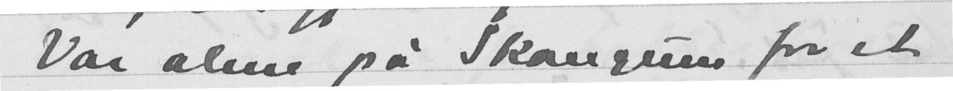Var alene på Skaugum for et
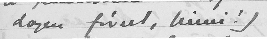dagen førset, Ninni!)
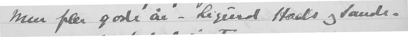Men blir gode år - Sigurd Hoels og Sande-
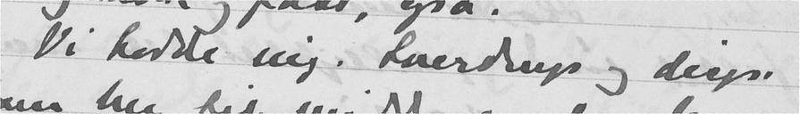Vi hadde mig, Sverdrups og disps.
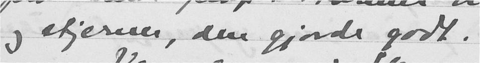og stjernerier, den gjorde godt.
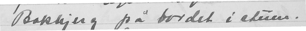Bokbjerg på bordet i stuen.
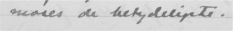moses de betydeligste.
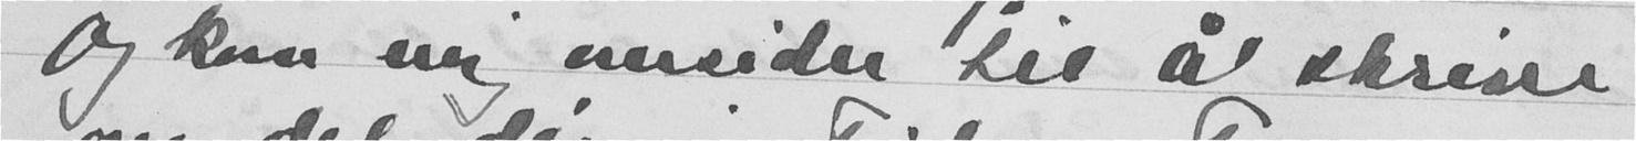Og kom mig omsider til å skrive
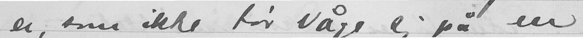er, som ikke tør våge seg på en
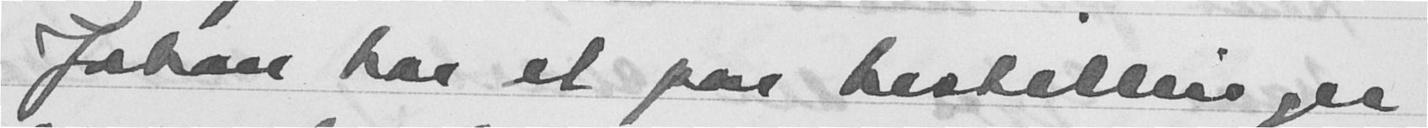Johan har et par bestillinger
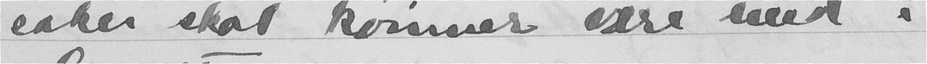saker skal kvinner være med i
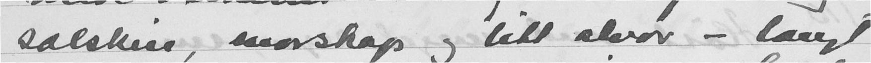solskin, morskap og litt alvor - langt
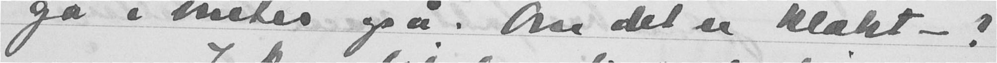gå i vinter også. Om det er klokt -?
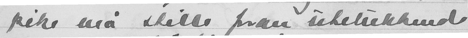pike må stille foran utelukkende
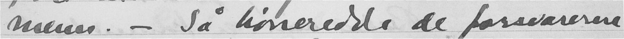menn. - Sa høneredde de forsvarerne
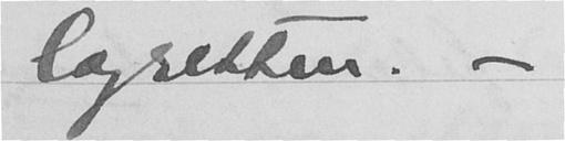lagretten. -
